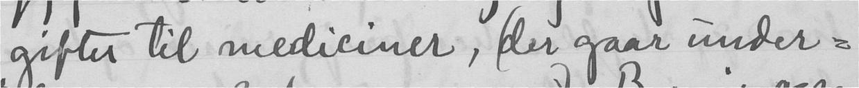gifter til mediciner, (der gaar under-
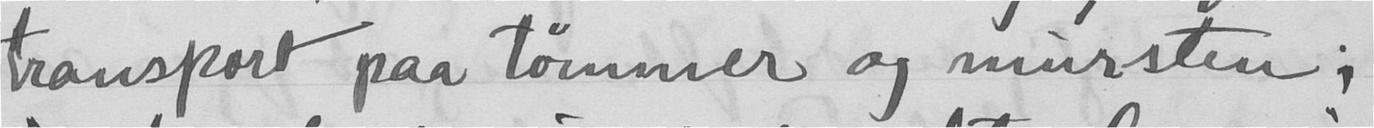transport paa tømmer og mursten;
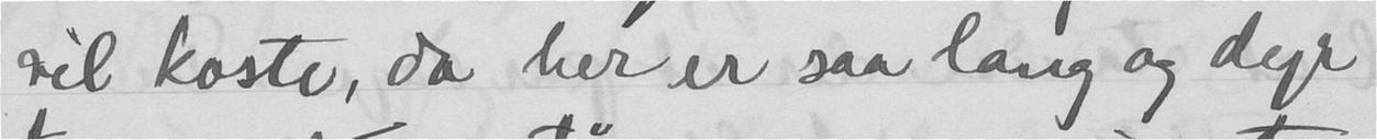vil koste, da her er saa lang og dyr
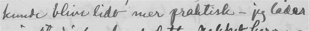kunde blive lidt mer prahtisk - jeg lader
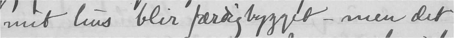mit hus blir færdigbygget - men det
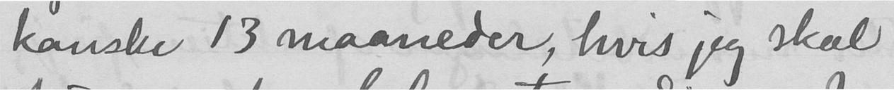kanske 13 maaneder, hvis jeg skal
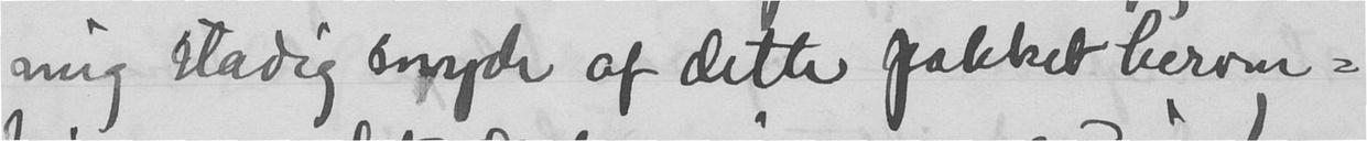mig stadig snyde af dette pakket herom-
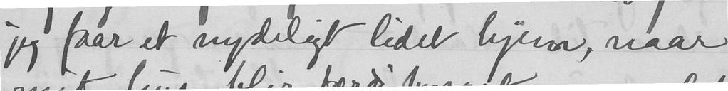jeg faar et nydeligt lidet hjem, naar
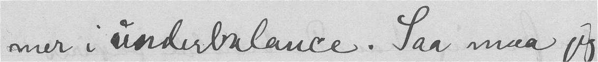mer i underbalance. Saa maa jeg
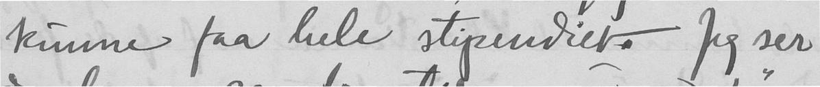kunne faa hele stipendiet. Jeg ser
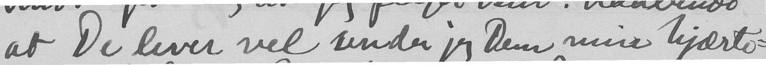at De lever vel under jeg dem min hjærte-
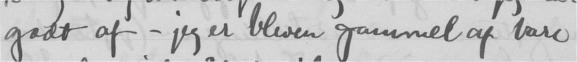godt af - jeg er bleven gammel af bare
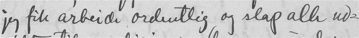jeg fik arbeide ordentlig og slap alle ud-
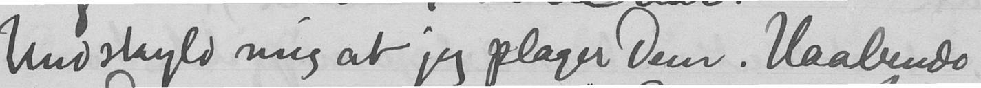Undskyld mig at jeg plager Dem. Haabende
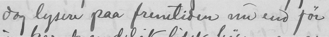dog lysere paa fremtiden nu end før
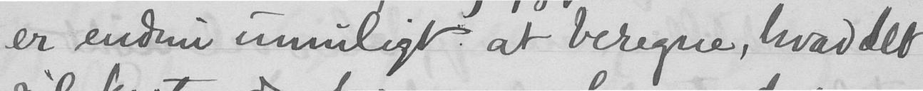er endnu umuligt at beregne, hvad det
